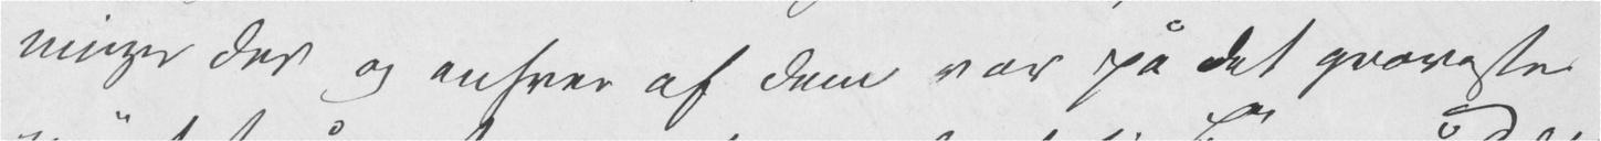ninger der og enhver af dem var på det groveste
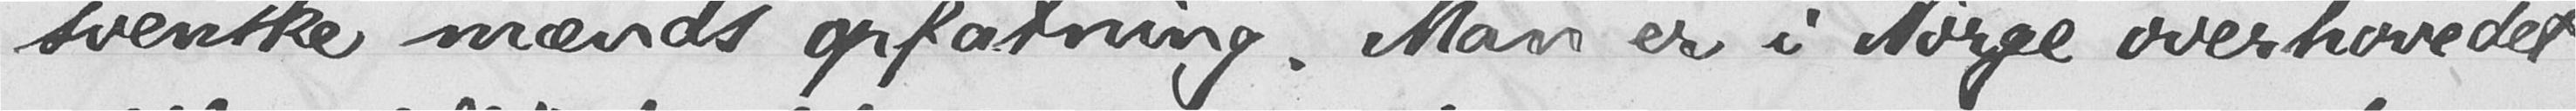svenske mænds opfatning. Man er i Norge overhovedet
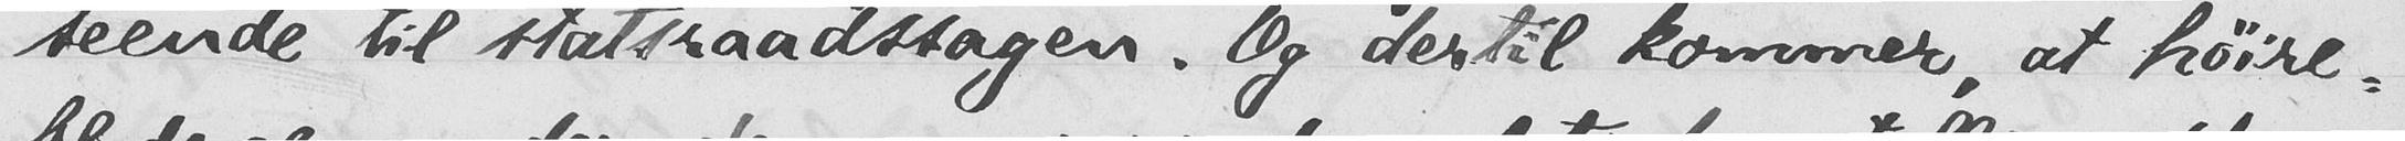seende til statsraadssagen. Og dertil kommer, at høire-
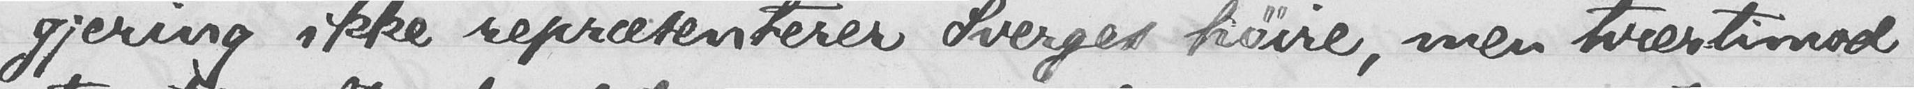gjering ikke repræsenterer Sverges høire, men tværtimod
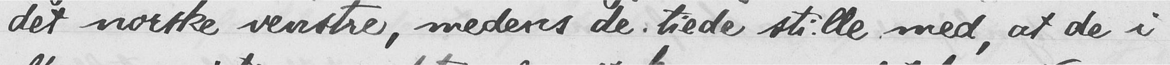det norske venstre, medens de tiede stille med, at de i
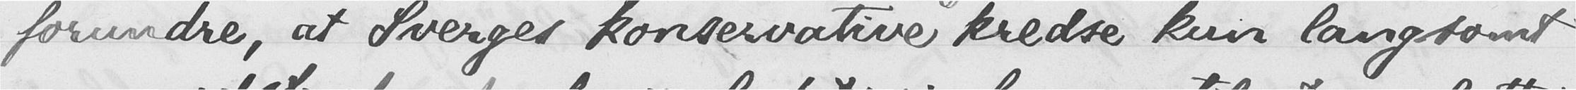forundre, at Sverges konservative kredse kun langsomt
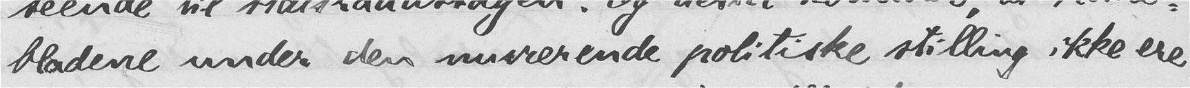bladene under den nuværende politiske stilling ikke ere
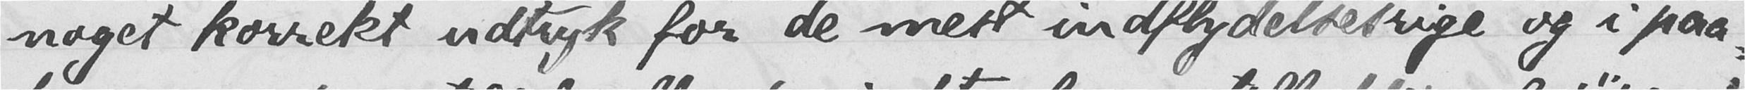noget korrekt udtryk for de mest indflydelsesrige og i paa-
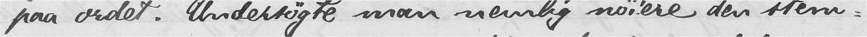paa ordet. Undersøgte man nemlig nøiere den stem-
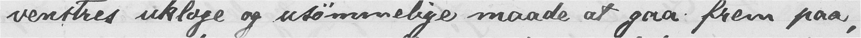venstres ukloge og usømmelige maade at gaa frem paa,
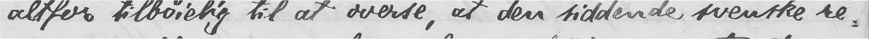altfor tilbøielig til at overse, at den siddende svenske re-
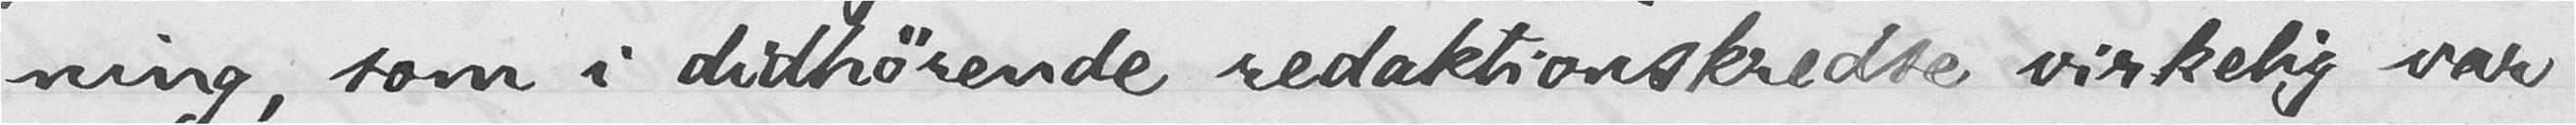ning, som i didhørende redaktionskredse virkelig var
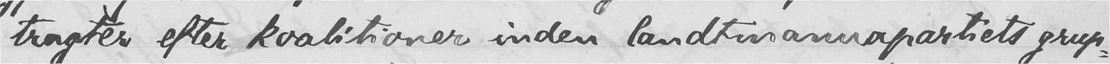tragter efter koalitioner inden landtmannapartiets grup-
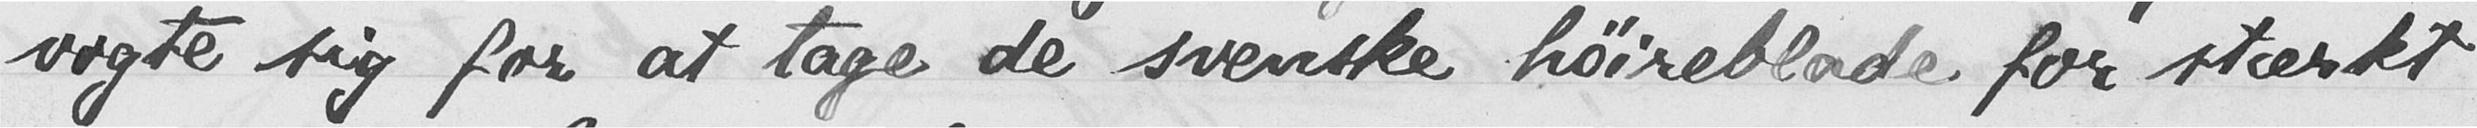vogte sig for at tage de svenske høireblade for stærkt
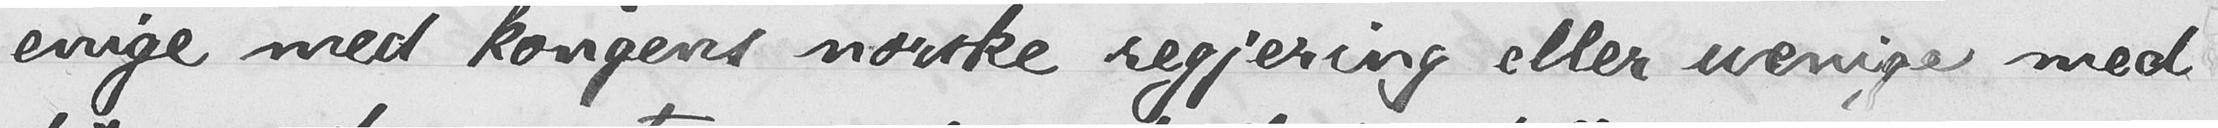enige med kongens norske regjering eller uenige med
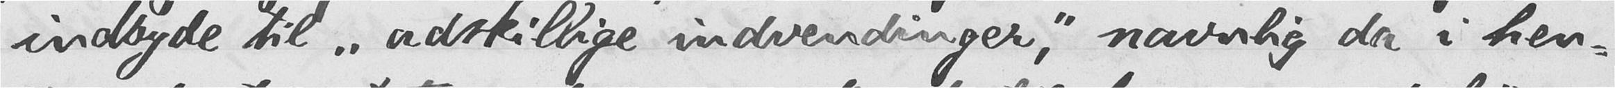indbyde til "adskillige indvendinger", navnlig da i hen-
